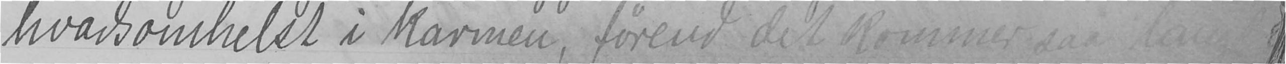hvadsomhelst i karmen, førend det kommer saa langt
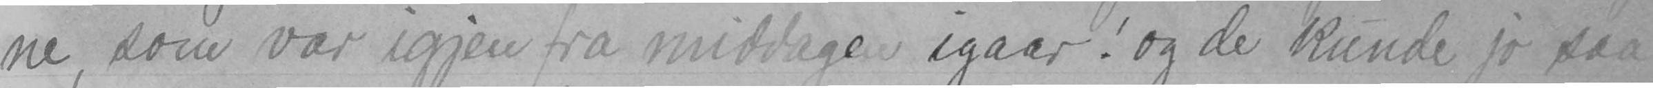ne, som var igjen fra middagen igaar! og de kunde jo saa
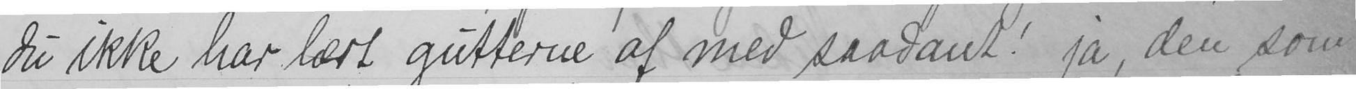du ikke har lært gutterne af med saadant! ja, den som
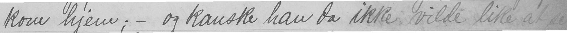kom hjem; - og kanske han da ikke vilde like at se
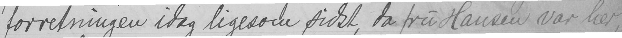forretningen idag ligesom sidst da fru Hansen var her,
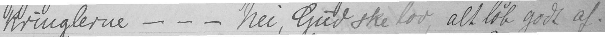kringlerne - - - nei, Gudskelov, alt løb godt af.
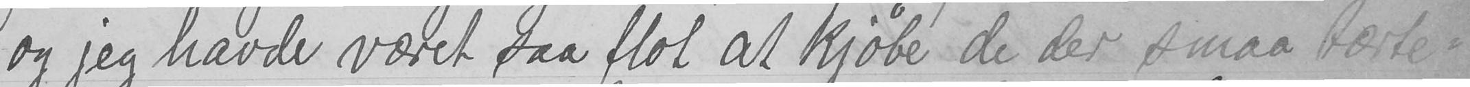og jeg havde været saa flot at kjøbe de der smaa tærte-
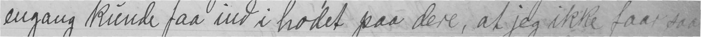engang kunde faa ind i hodet paa dere, at jeg ikke faar saa
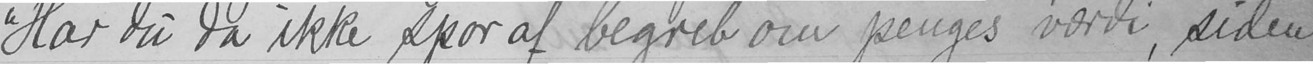"Har du da ikke spor af begreb om penges verdi, siden
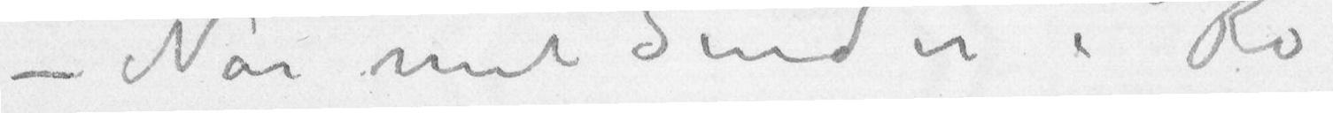– Når mit Sind er i Ro
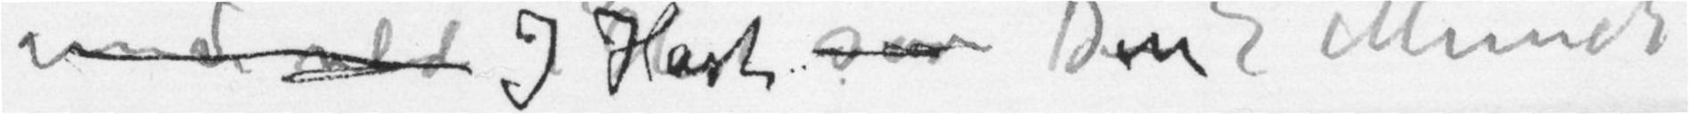I HastDin E Munch
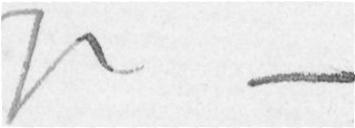op –
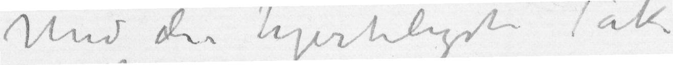Med den hjerteligste Tak
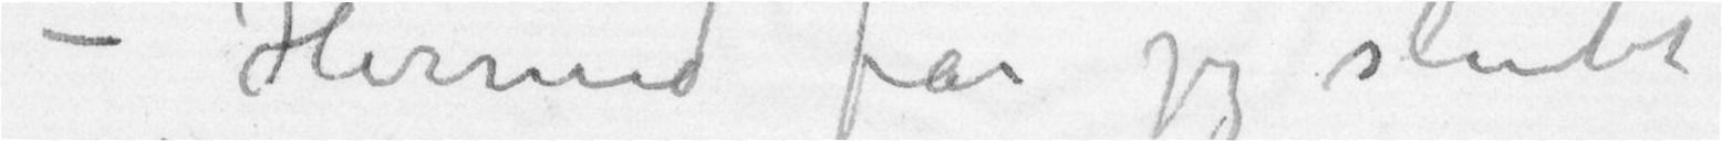– Hermed får jeg slutte
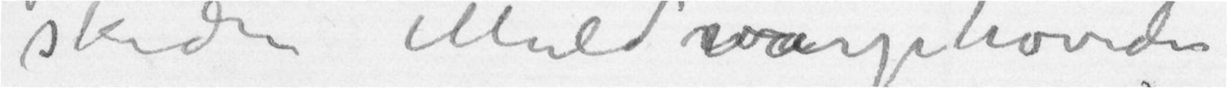skidne Muldvarphoveder
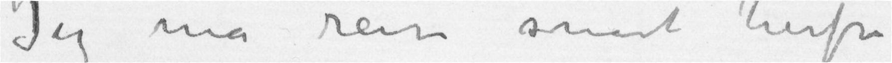Jeg må reise snart herfra
